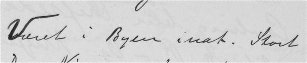Været i Byen inat. Stort
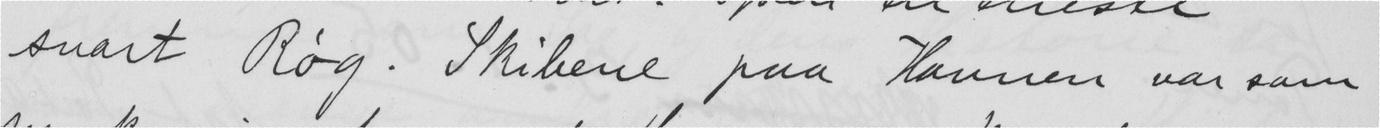svart Røg. Skibene paa Havnen var som
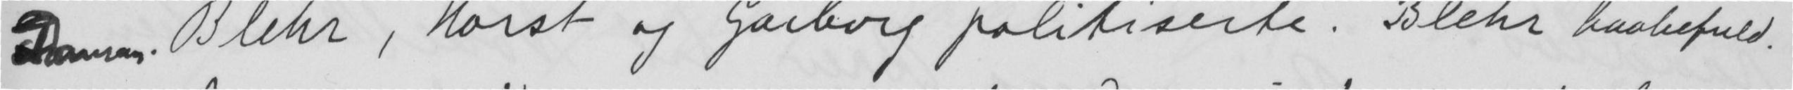Dansen. Blehr, Horst og Garborg politiserte. Blehr haabefuld.
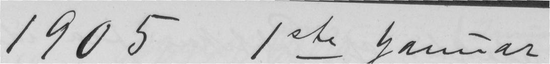1905 1ste Januar
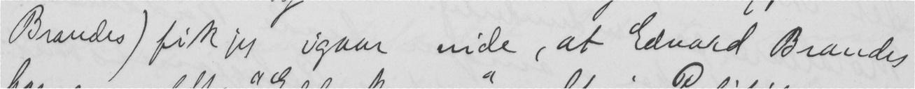Brandes) fik jeg igaar vide, at Edvard Brandes
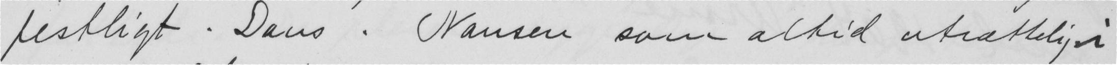festligt. Dans. Nansen som altid utrættelig i
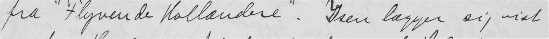fra "Flyvende Hollændere". Isen lægger sig vist
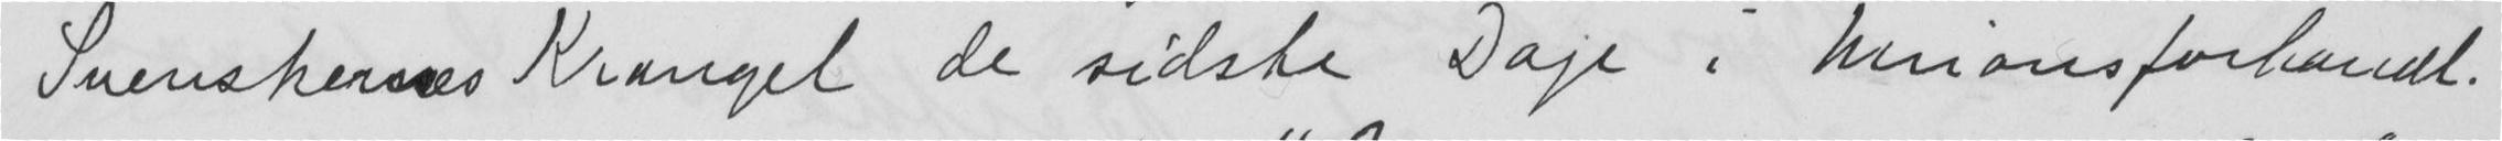Svenskerns Krangel de sidste Dage i Unionsforhandl.
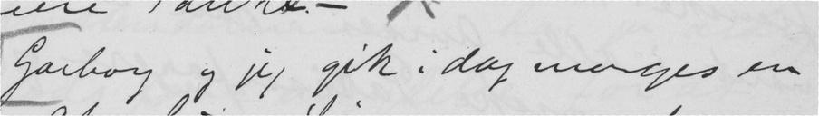Garborg i jeg gik i dag morges en
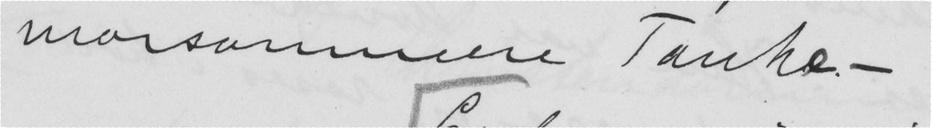morsommere Tanke -
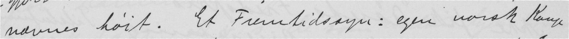nævnes høit. Et Fremtidssyn: egen norsk Konge
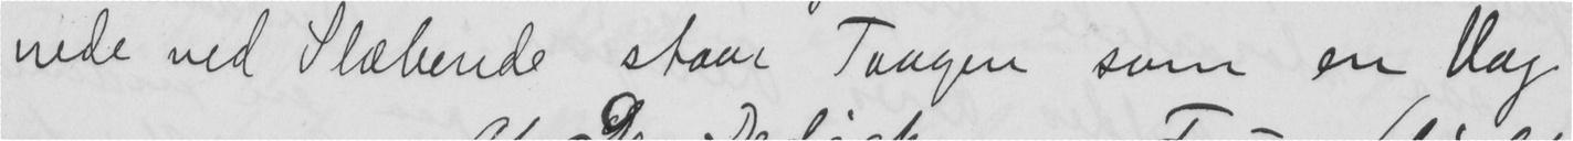nede ved Slæbende staar Taagen som en Vag
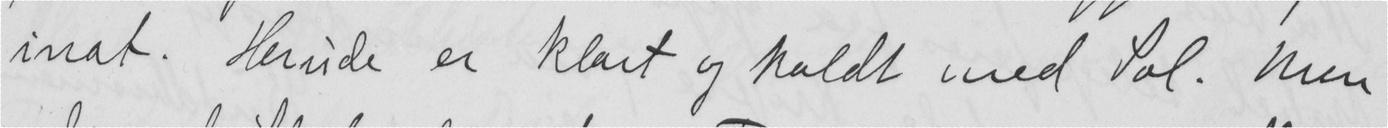inat. Herude er klart og kaldt med Sol. Men
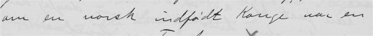om en norsk indfødt Konge var en
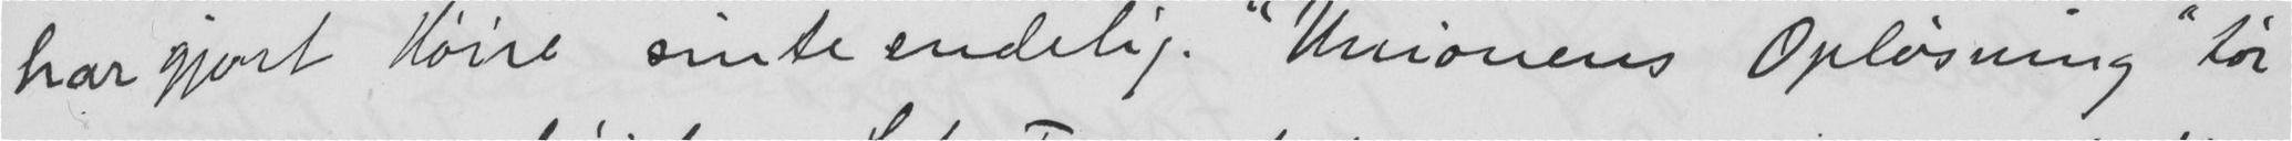har gjort Høire sinte endelig. "Unionens Opløsning" tør
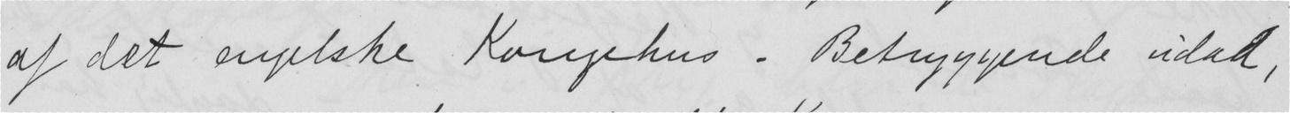af det engelske Kongehus. Betryggende udad,
